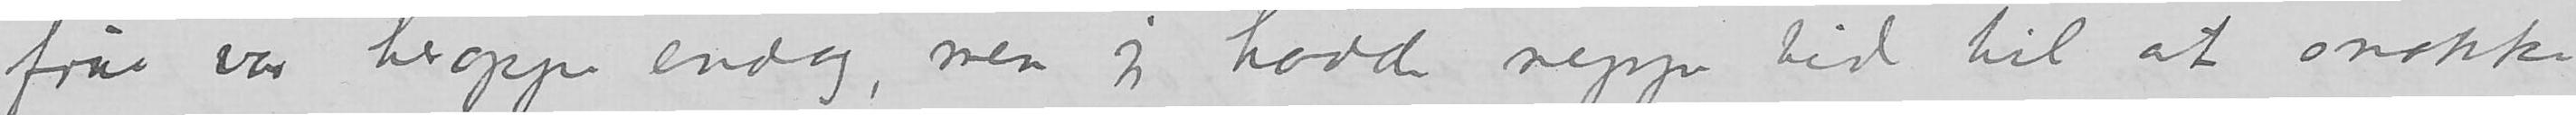frue var heroppe endag, men jeg hadde neppe tid til at snakke
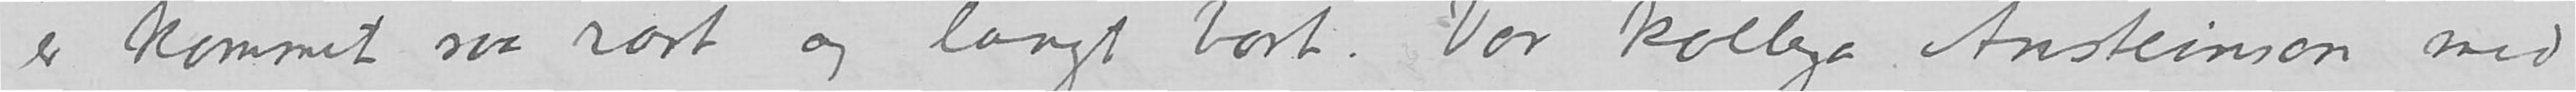er kommet saa rart og langt borte. Vor kollega Ansteinson med
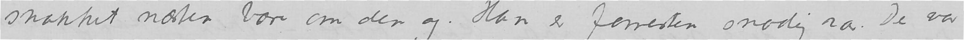snakket næsten bare om den og. Han er forresten snodig rar. De var
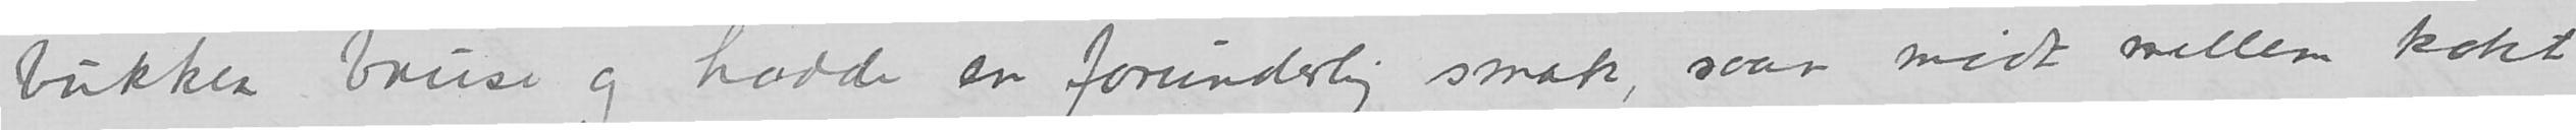bukken bruse og hadde en forunderlig smak, saan midt mellem kokt
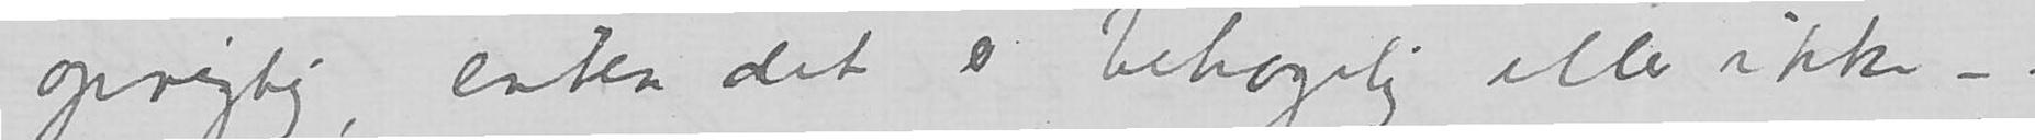oprigtig, enten det er behagelig eller ikke –.
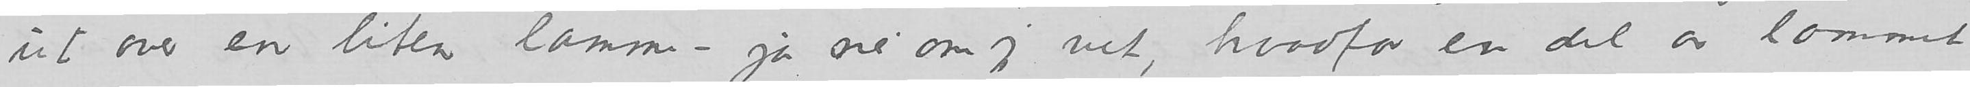ut over en liten lamm – ja nei om jeg vet, hvadfor en del av lammet
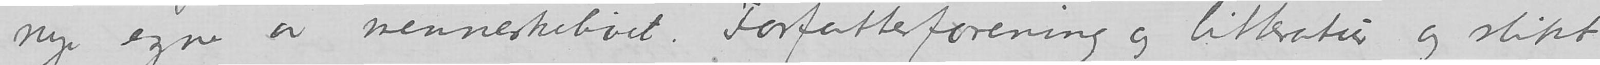nye egne av menneskelivet. Forfatterforening og litteratur og slikt
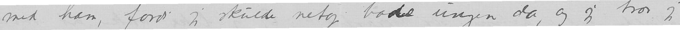med ham, fordi jeg skulde netop bade ungen da, og jeg tror jeg
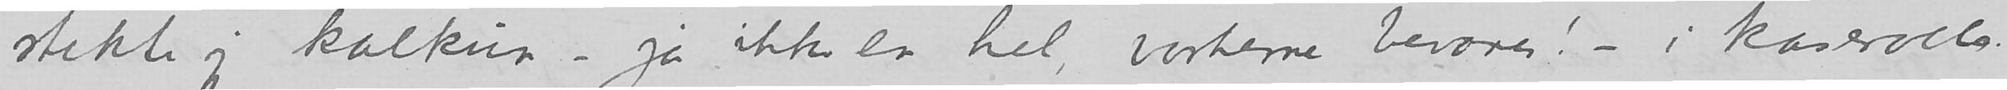stekte jeg kalkun – ja ikke en hel, vorherre bevares! – i kaserolle.
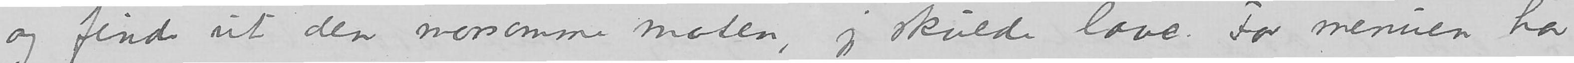og finde ut den morsomme maten, jeg skulde lave. For menuen har
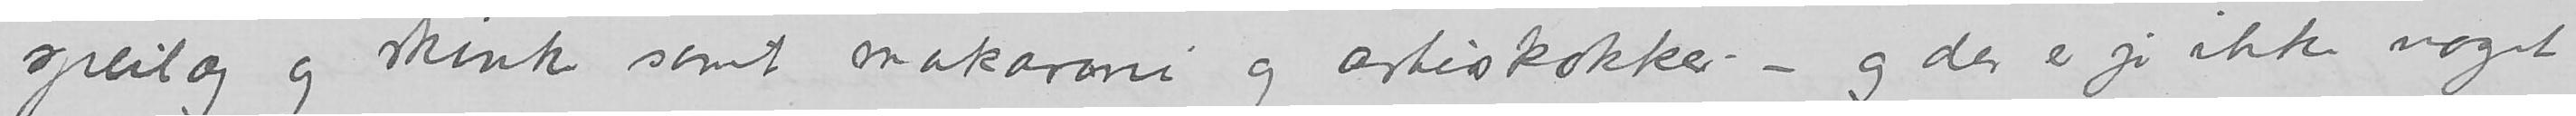speilæg og skinke samt makaroni og artiskokker – og der er jo ikke noget
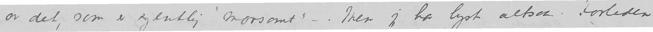av det, som er egentlig «morsomt» –. Men jeg har lyst altsaa. Forleden
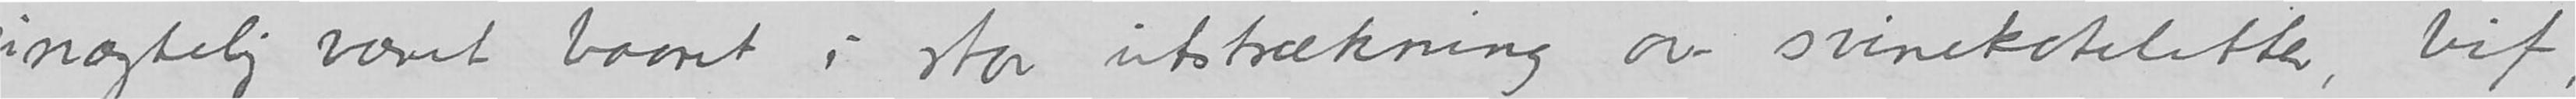unægtelig været baaret i stor utstrækning av svinekoteletter, bif,
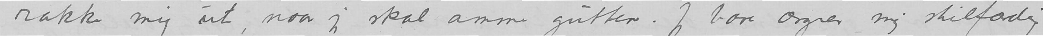rakke mig ut, naar jeg skal amme gutten. Jeg bare ærgrer mig stilfærdig
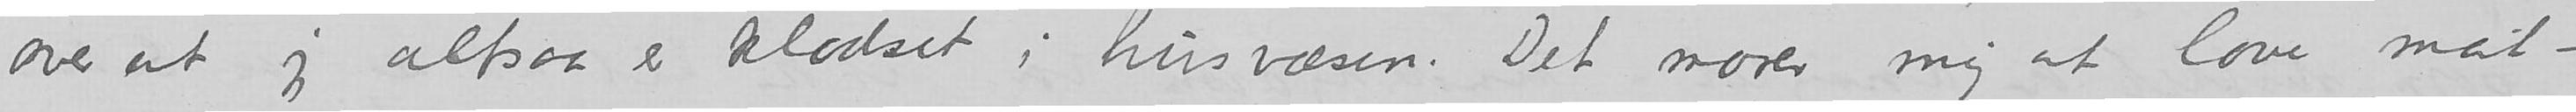over at jeg altsaa er klodset i husvæsen. Det morer mig at lave mat –
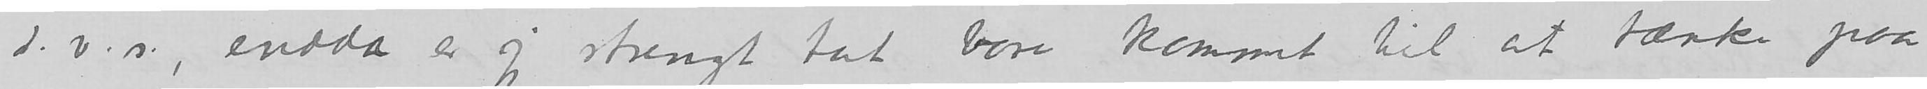d.v.s., endda er jeg strengt tat bare kommet til at tænke paa
